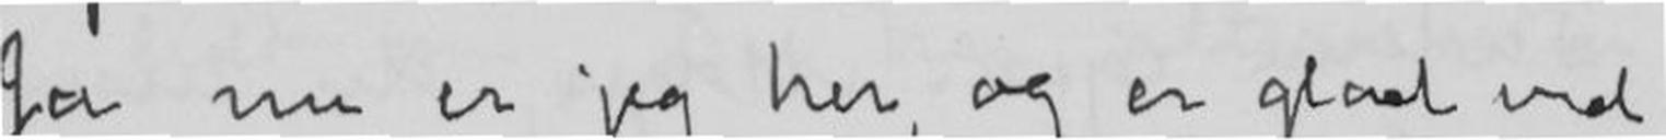Ja nu er jeg her, og er glad ved
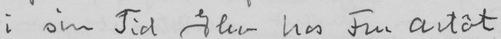i sin Tid Elev hos Fru Artôt
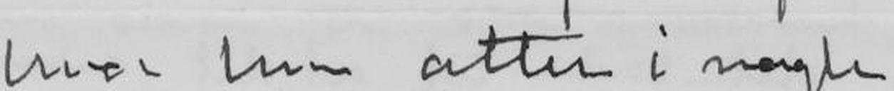hvor han atter i nogle
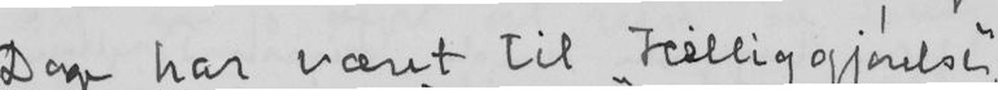Dage har været til "Helliggjørelse",
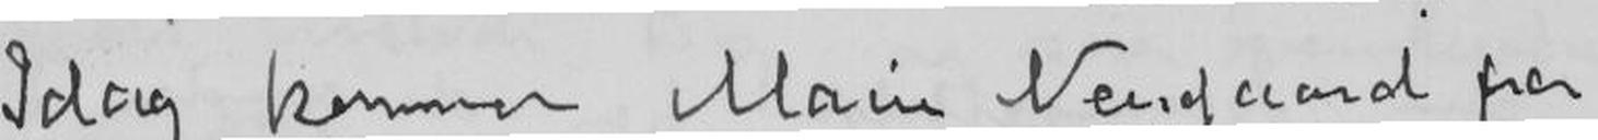Idag kommer Main Neuqaard fra
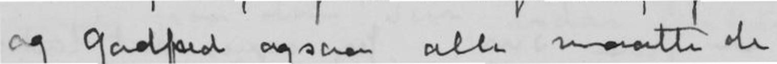og Godfred ogsaa alle maatte de
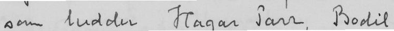som hedder Hagar Pass, Bodil
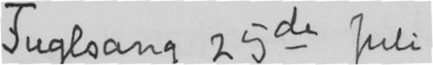Fuglsang 25de Juli
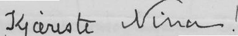Kjæreste Nina!
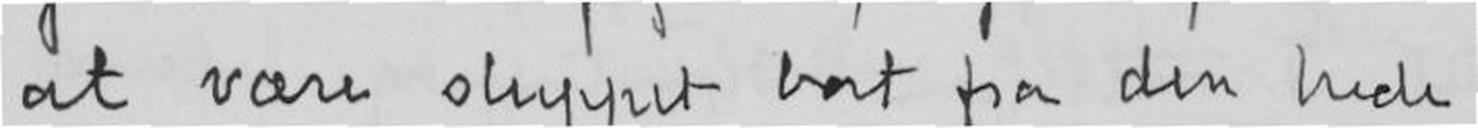at være sluppet bort fra den hede
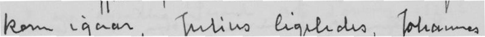kom igaar, Julius ligeledes, Johannes
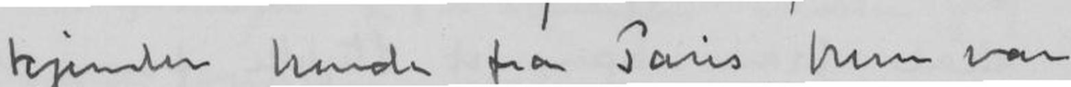kjender hende fra Paris hun var
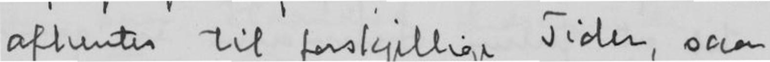afluntes til forskjellige Tider, saa
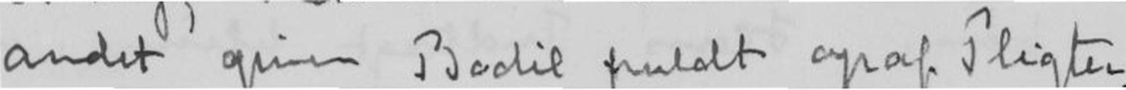andet giver Bodil fuldt opaf Pligter.
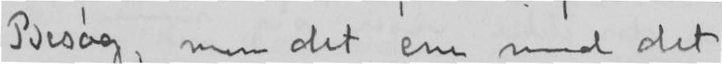besøg, men det ene med det
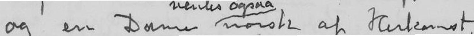og en Dame norsk af Herkomst,
